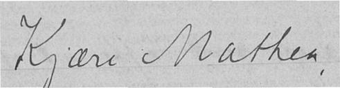Kjære Mathea,
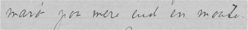marø paa mere end en maate.
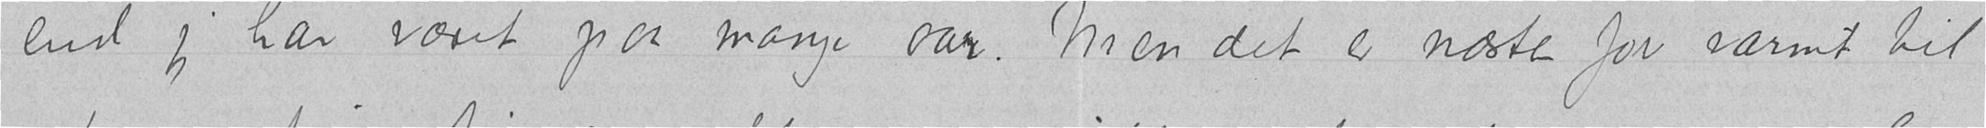end jeg har været paa mange aar. Men det er næsten for varmt til
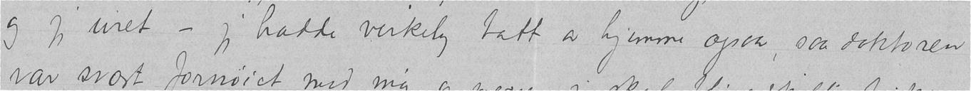og jeg uret – jeg hadde virkelig tatt av hjemme ogsaa, saa doktoren
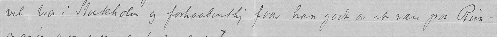vel bra i Stockholm og forhaabentlig faar han godt av at være paa Run-
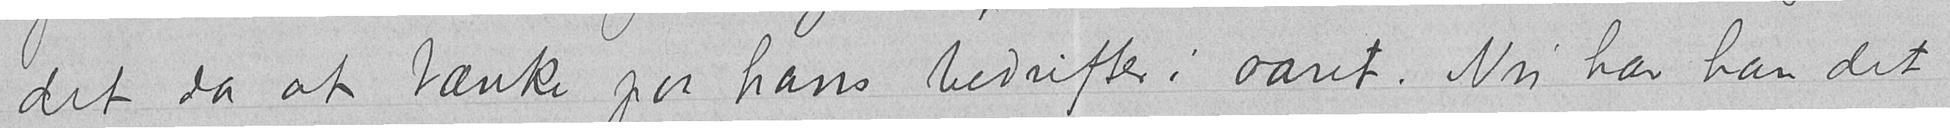det da at tænke paa hans bedrifter i aaret. Nu har han det
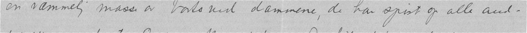en væmmelig masse av borte ved dammene, de har spist op alle and-
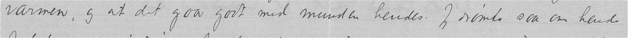varmen, og at det gaar godt med munden hendes. Jeg drømte saa om hende
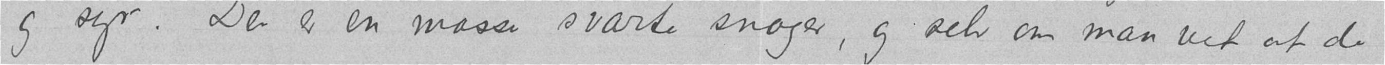og syr. Der er en masse svarte snoger, og selv om man vet at de
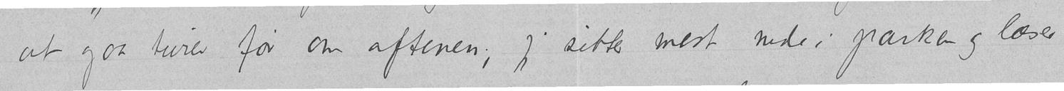at gaa turer før om aftenen, jeg sitter mest nede i parken og læser
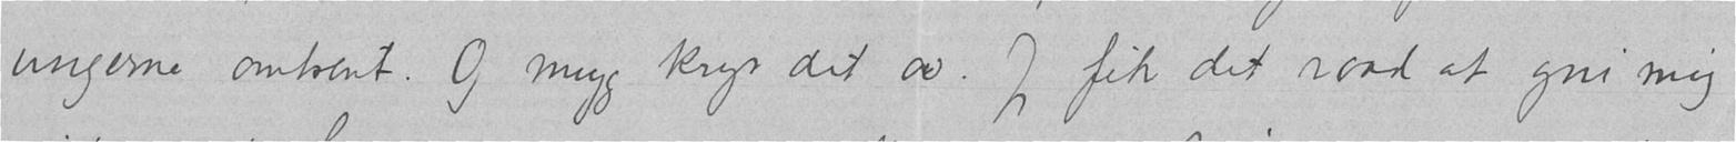ungerne omtrent. Og myg kryr det av. Jeg fik det raad at gni mig
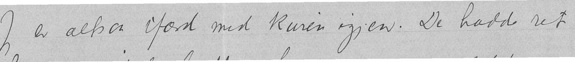Jeg er altsaa ifærd med kuren igjen. De hadde ret
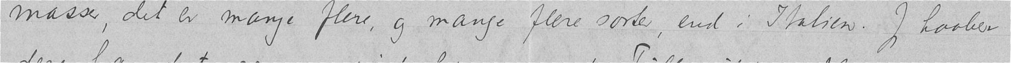masser, det er mange flere, og mange flere sorter, end i Italien. Jeg haaber
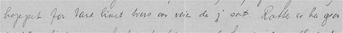hoppet for bare livet tvers over veien der jeg sat. Rotter er her ogsaa
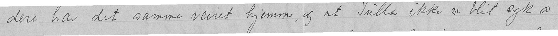dere har det samme veiret hjemme, og at Tulla ikke er blit syk av
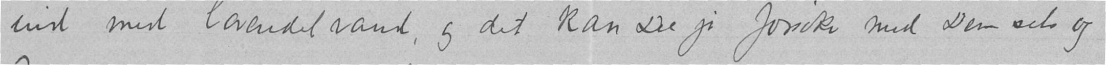ind med lavendelvand, og det kan De jo forsøke med Dem selv og
